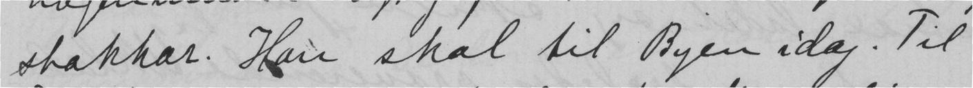stakkar. Han skal til Byen idag. Til
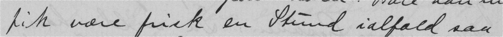fik være frisk en Stund ialfald saa
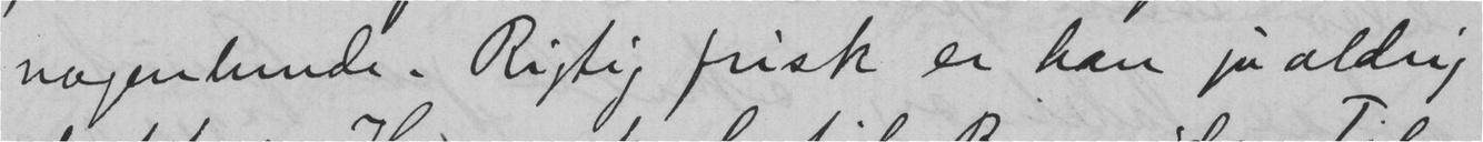nogenlunde. Rigtig frisk er han jo aldrig
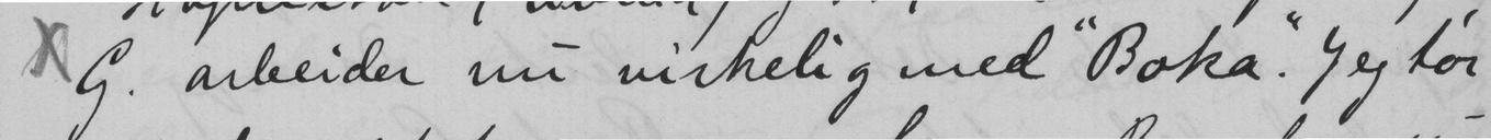x G. arbeider nu virkelig med "Boka". Jeg tør
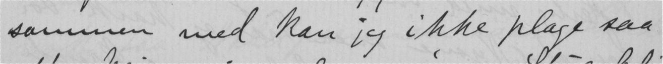sammen med kan jeg ikke plage saa
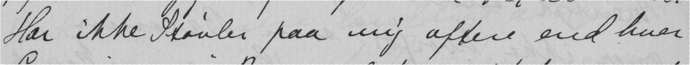Har ikke støvler paa mig oftere end hver
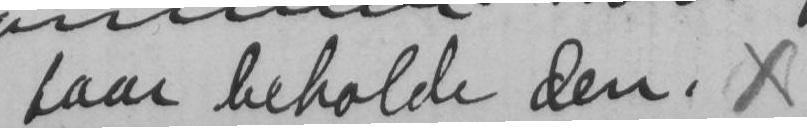faar beholde den. x
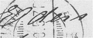Eders
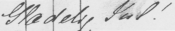Glædelig Jul!
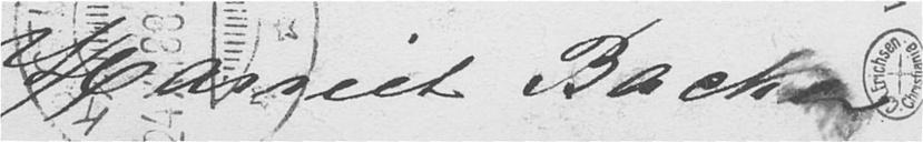Harriet Backer
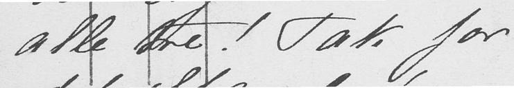alle tre! Tak for
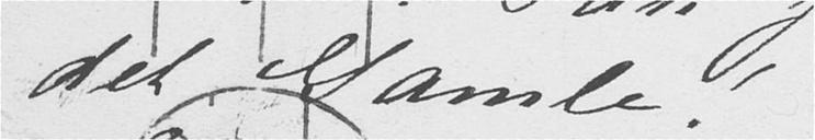det Gamle!
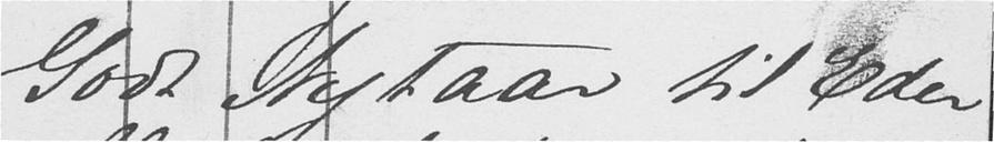Godt Nytaar til Eder
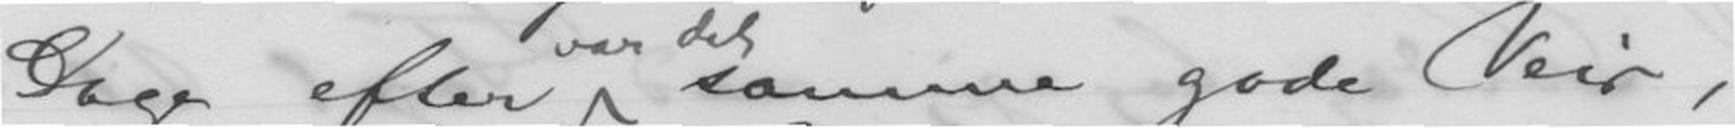Dage efter samme gode Veir,
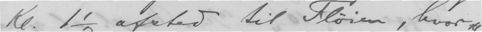l. 1½ afsted til Fløien, hvor
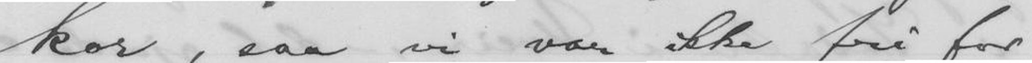kor, saa vi var ikke fri for
